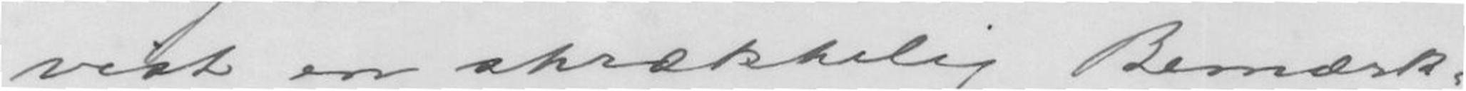vist en skrækkelig Bemærk-
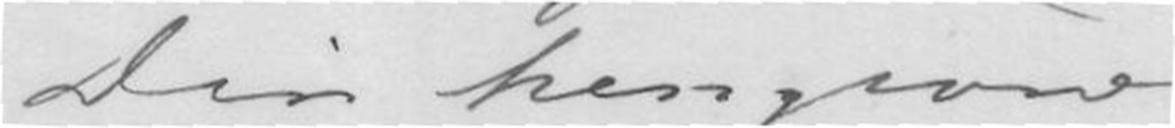Din hengivne
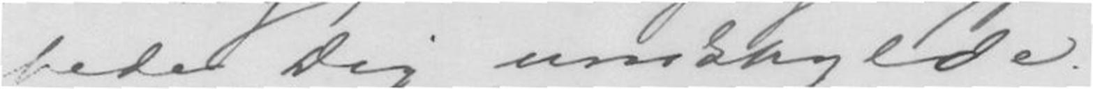bede Dig undskylde.
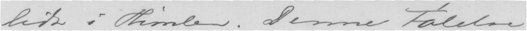lidt i Himlen. Denne Følelse
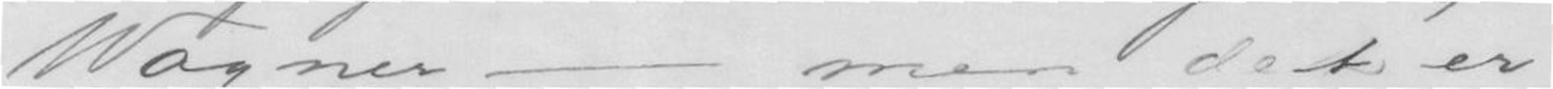Wagner---- men det er
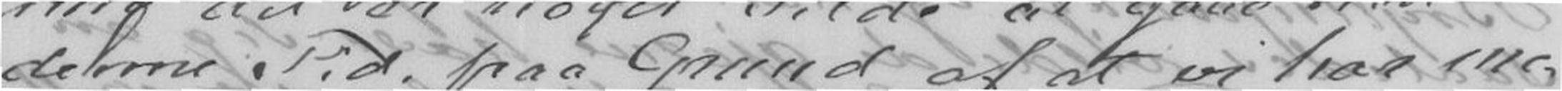denne Tid paa Grund af at vi har me-
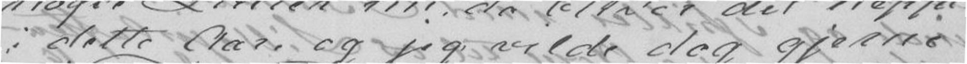i dette Aar, og jeg vilde dog gjerne
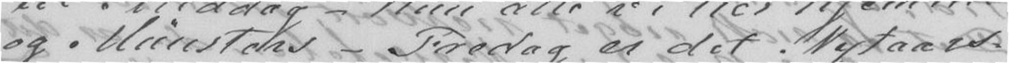og Münsters - Fredag er det Nytaars-
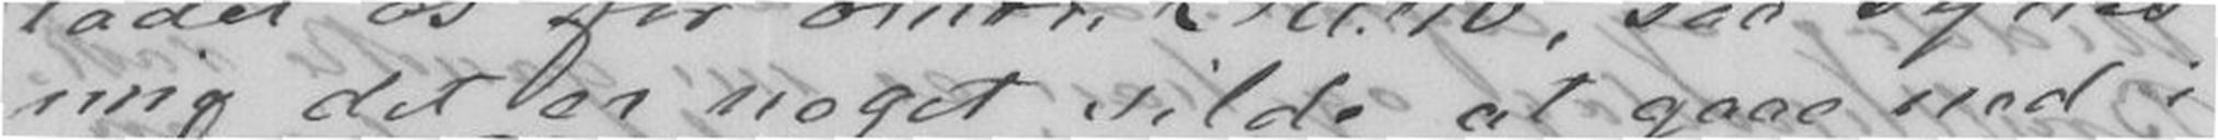mig det er noget silde at gaae ned i
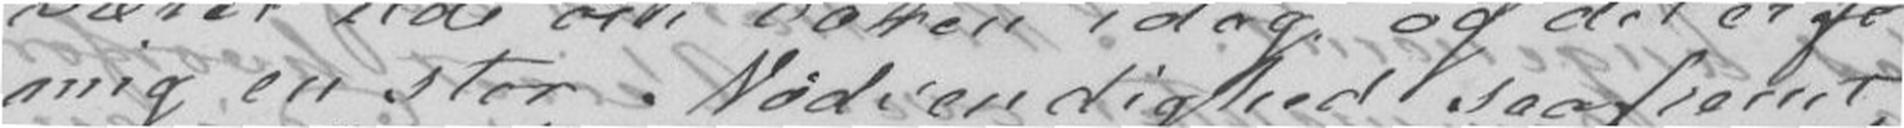mig en stor Nødvendighed saafremt
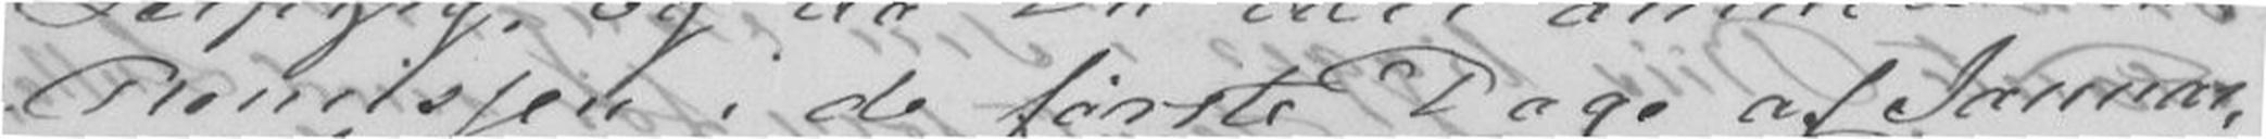Renisjen i de første Dage af Januar
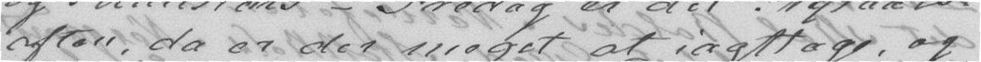aften, da er der meget at iagtage, og
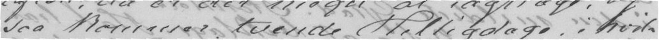saa kommer tvende Helligdage i hvil-
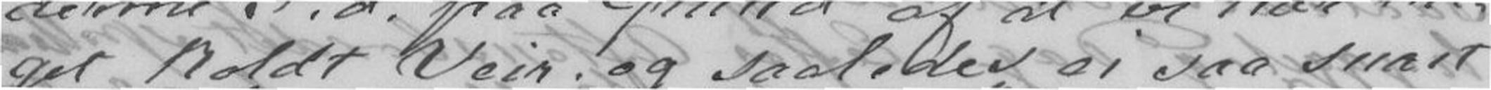get koldt Veir og saaledes ei saa snart
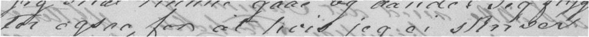ter ogsaa for at hvis jeg ei skriver
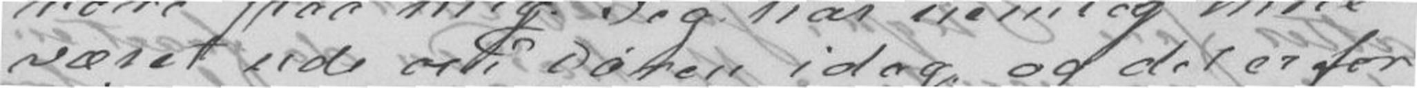været ude om Døren idag og det er for
